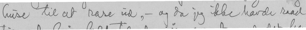huse til at rase ud, - og da jeg ikke havde raad
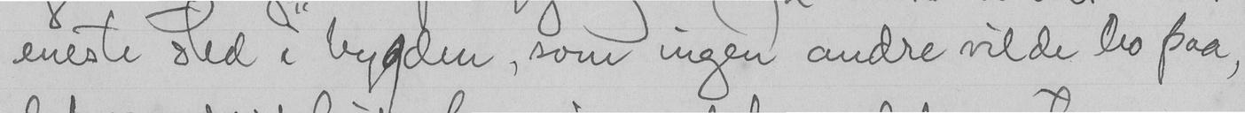eneste sted i bygden, som ingen andre vilde bo paa,
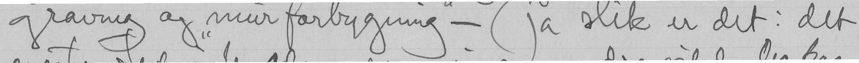graving og mur forbygning" - (ja slik er det: det
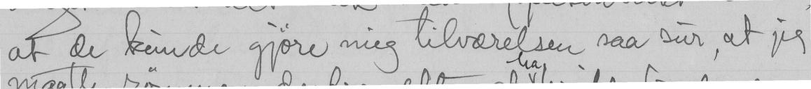at de kunde gjøre mig tilværelsen saa sur, at jeg
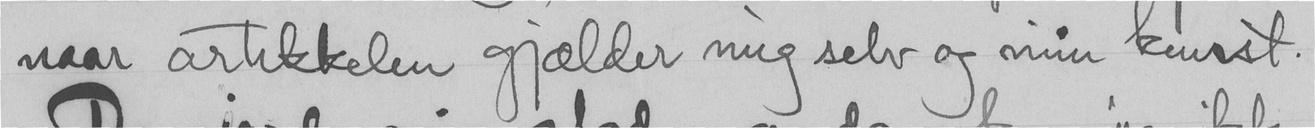naar artikkelen gjælder mig selv og min kunst.
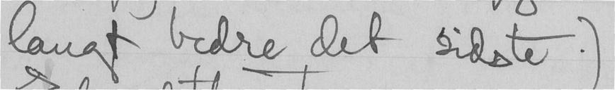langt bedre det sidste.)
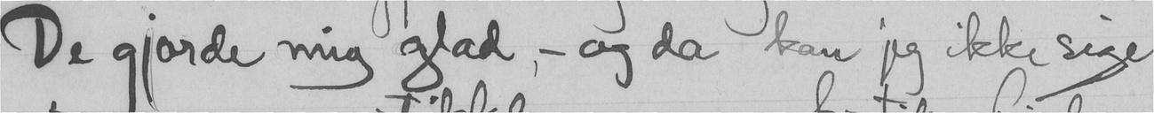De gjorde mig glad, - og da kan jeg ikke sige
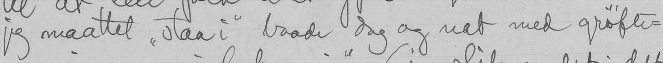jeg maatet "staa i" baade dag og nat med grøfte-
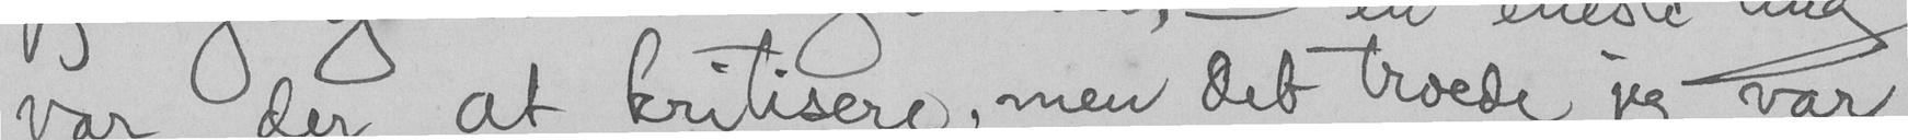var der at kritisere, men det troede jeg - var
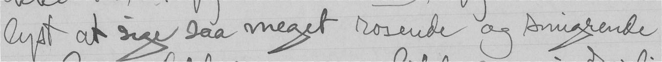lyst at sige saa meget rosende og smigrende
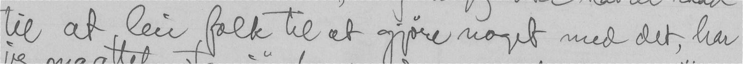til at leie folk til at gjøre noget med det, har
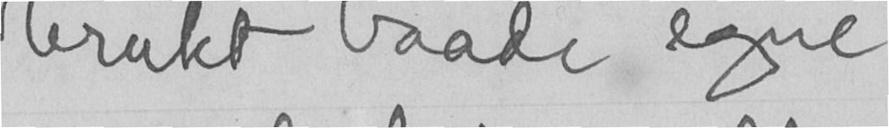brukt baade egne
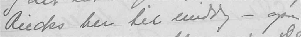Riecks her til middag - ogsaa
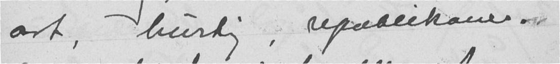art, hurtig, republikaner.
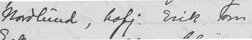Nordlund, hofj. Erik von
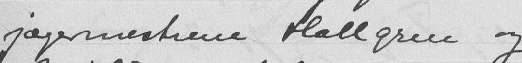jægermestrene Hallgren og
